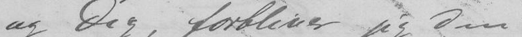og Dig, forbliver jeg Din
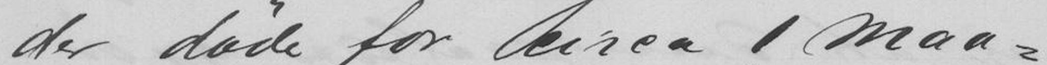der døde for circa 1 Maa-
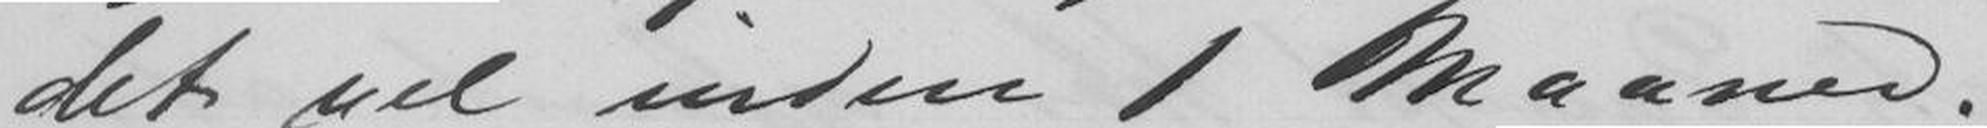det vel inden 1 Maaned. -
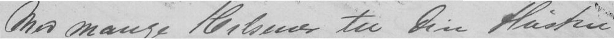Med mange Hilsener til Din Hustru
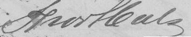Thor Hals
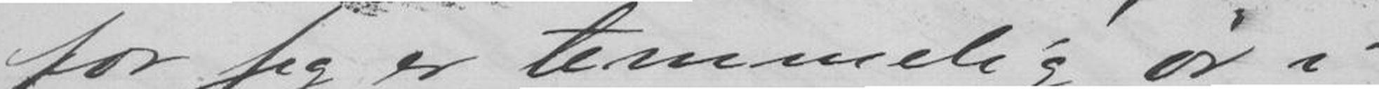for jeg er temmelig ør i
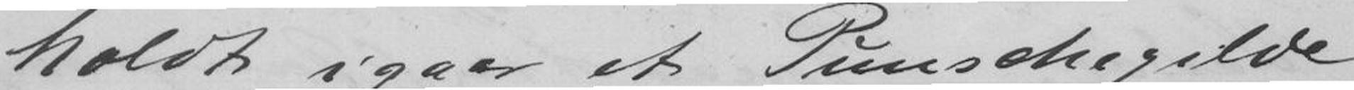holdt igaar et Punschegilde
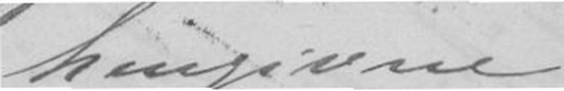hengivne
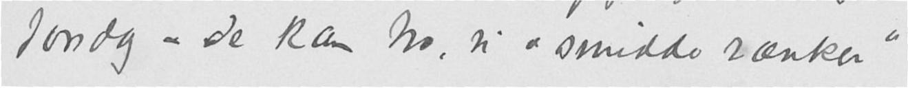torsdag – de kan tro, vi "smidde rænker"
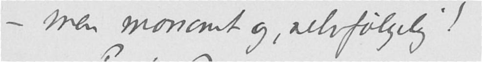– men morsomt og, selvfølgelig!
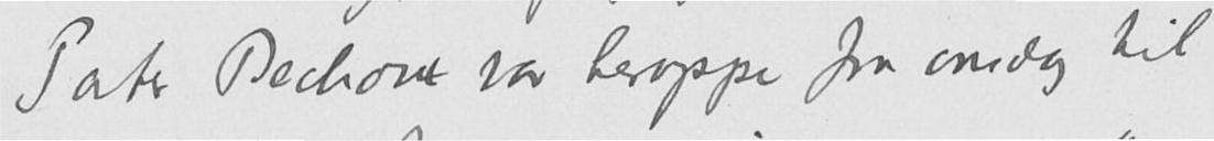Pater Bechaux var heroppe fra onsdag til
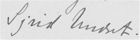Sigrid Undset.
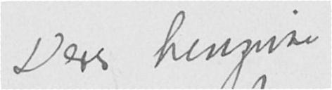Deres hengivne
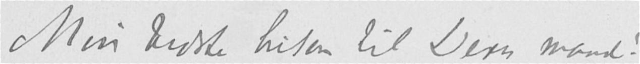Min bedste hilsen til Deres mand!
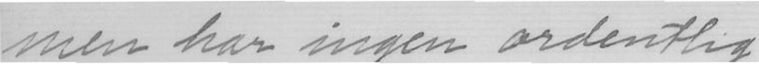men har ingen ordentlig
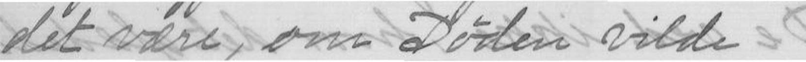det være, om Døden vilde
